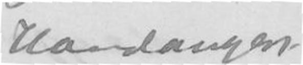Hardanger
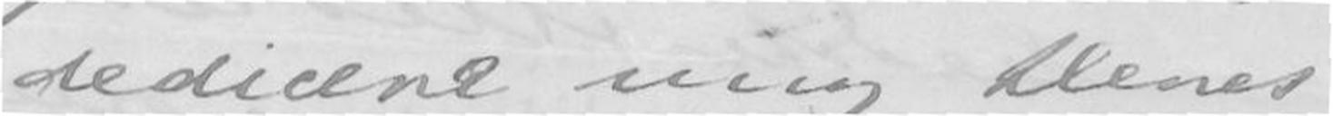dedicere mig Deres
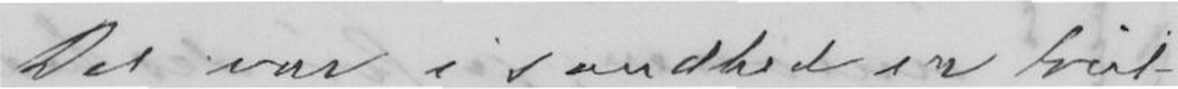Det var i sandhed trist
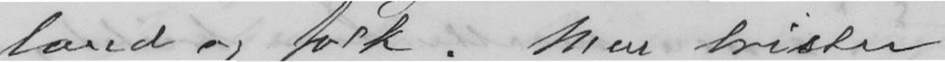land og folk. Men brister
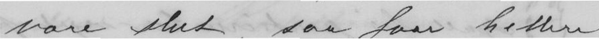være slut, saa faar hellere
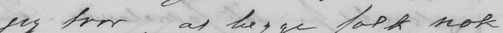jeg tror, at begge folk nok
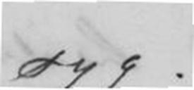syg.
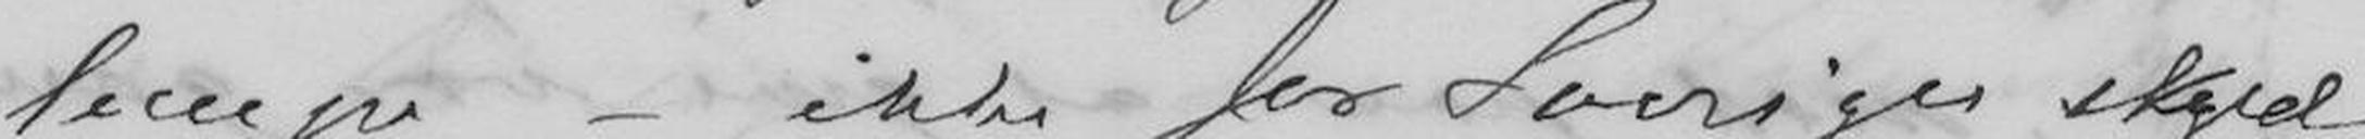lempe, - ikke for Sveriges skyld,
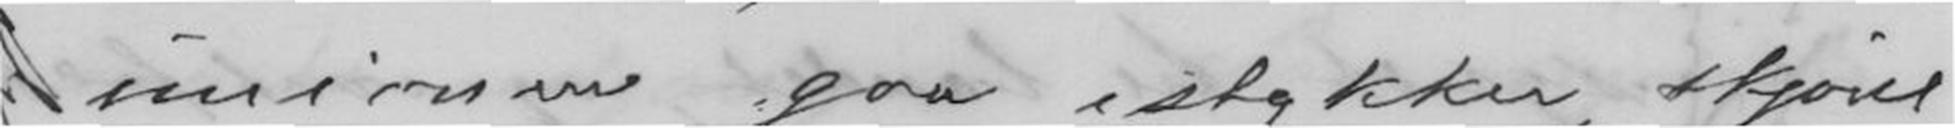unionen gaa istykker, skjønt
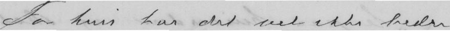For hun har det vel ikke bedre
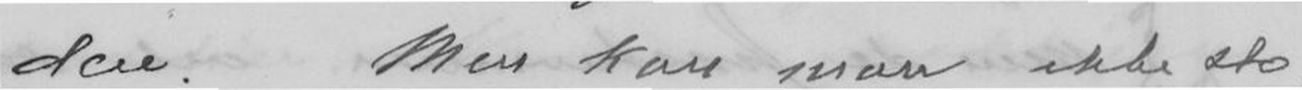den. Men kan man ikke sto-
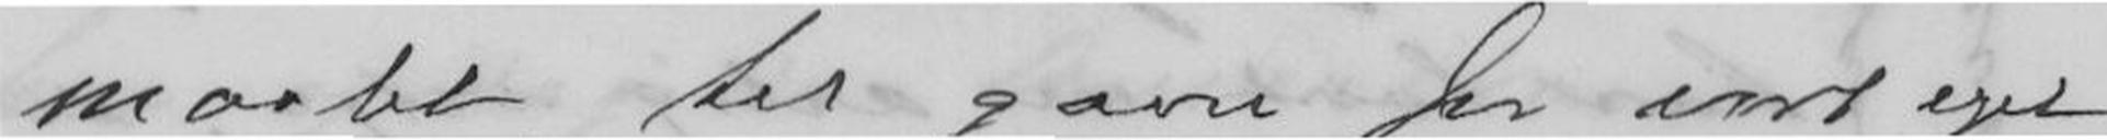maalet, til gavn for vort eget
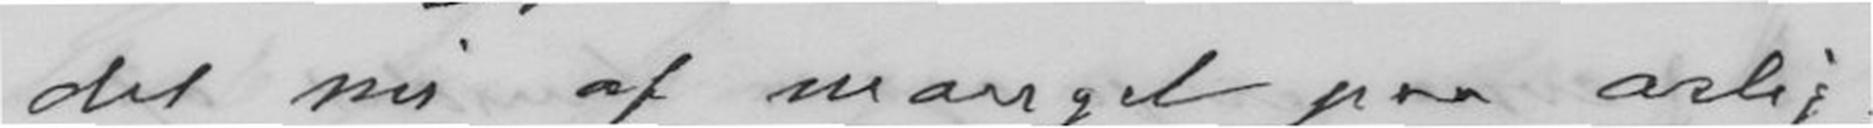det nu af mangel paa ærlig-
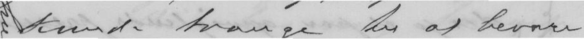kunde trænge til at bevare
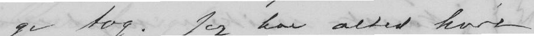ge tog. Jeg har altid hørt
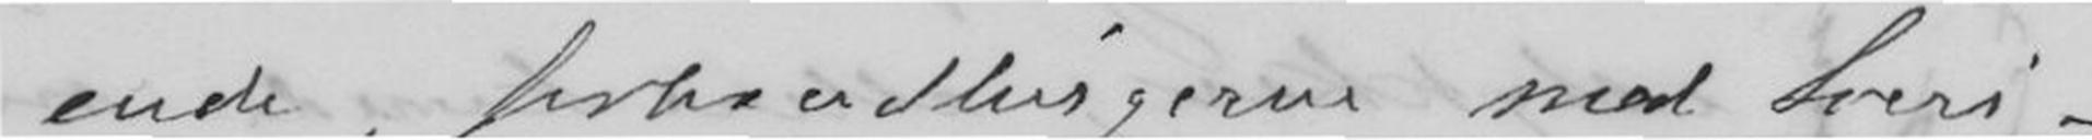ende, forhandlingerne med Sveri-
